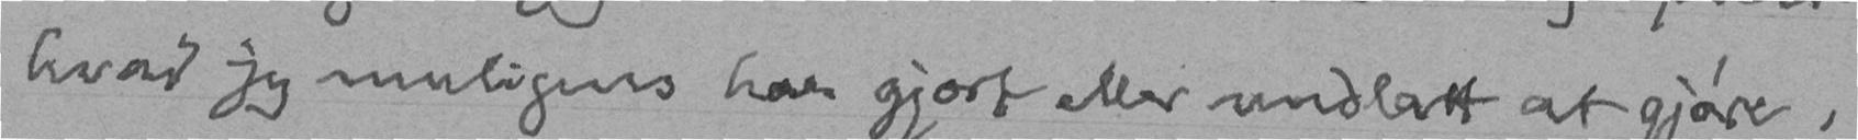hvad jeg muligens har gjort eller undlatt at gjøre,
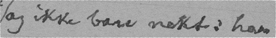og ikke bare nekt: har
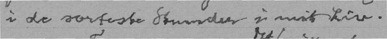i de sorteste Stunder i mit Liv.
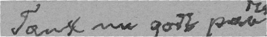Tænk nu godt paa
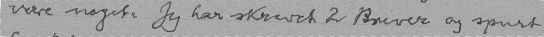være noget. Jeg har skrevet 2 Brever og spurt
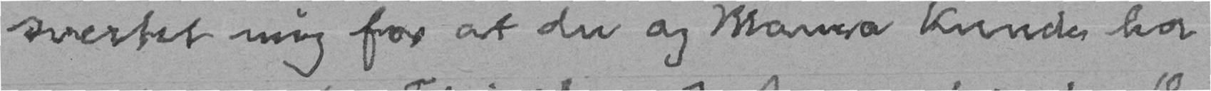svertet mig for at du og Mama kunde ha
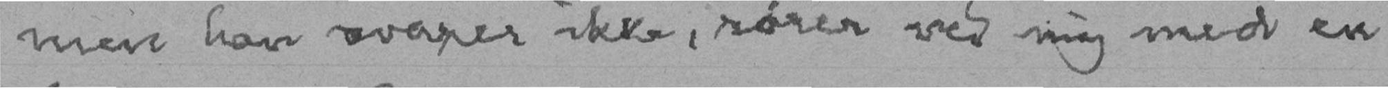men han svarer ikke, rører ved mig med en
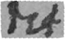det
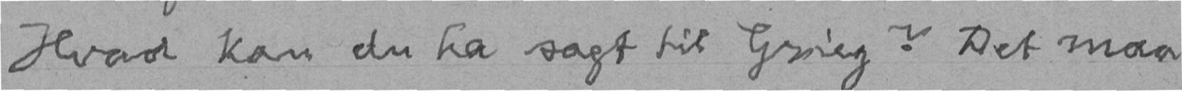Hvad kan du ha sagt til Grieg? Det maa
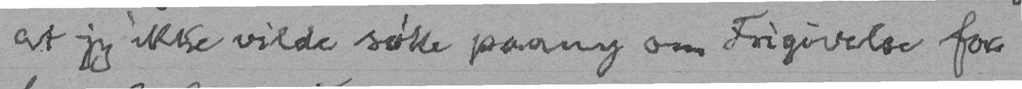at jeg ikke vilde søke paany om Frigivelse for
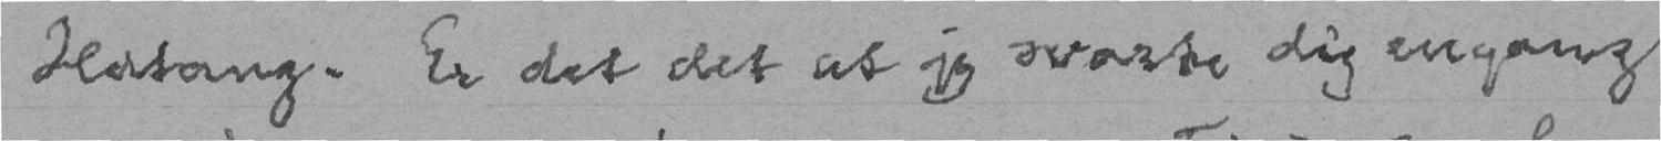Ildtang. Er det det at jeg svarte dig engang
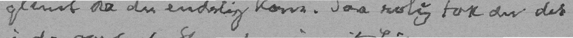glemt da du endelig kom. Saa rolig tok du det
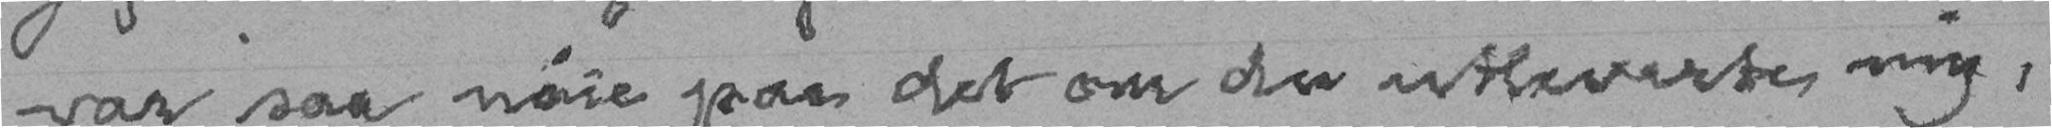var saa nøie paa det om du utleverte mig,
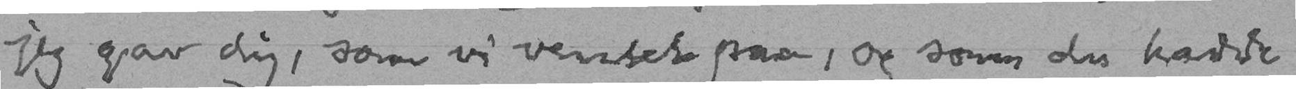jeg gav dig, som vi ventet paa, og som du hadde
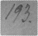193.
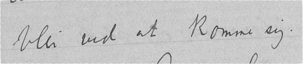blir ved at komme sig.
